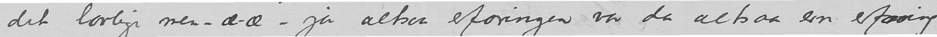kurat det lovlige men - æ-æ - ja altsaa erfaringen var da altsaa en erfaring
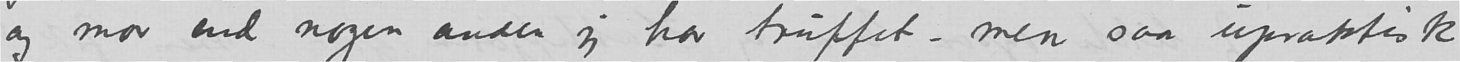og mor end nogen anden jeg har truffet - men saa upraktisk
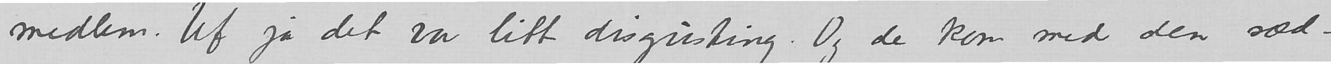medlem. Uf ja det var litt disgusting. Og de kom med den sæd-
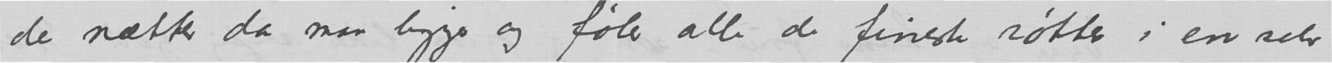om de nætter da man ligger og føler alle de fineste røtter i en selv
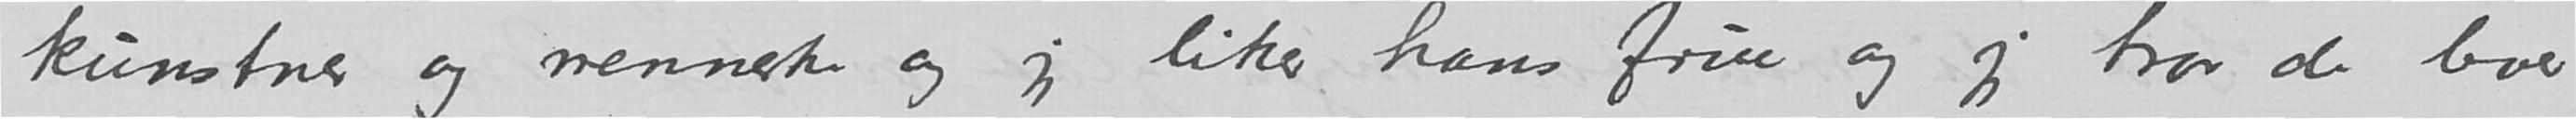kunstner og menneske og jeg liker hans frue og jeg tror de bor
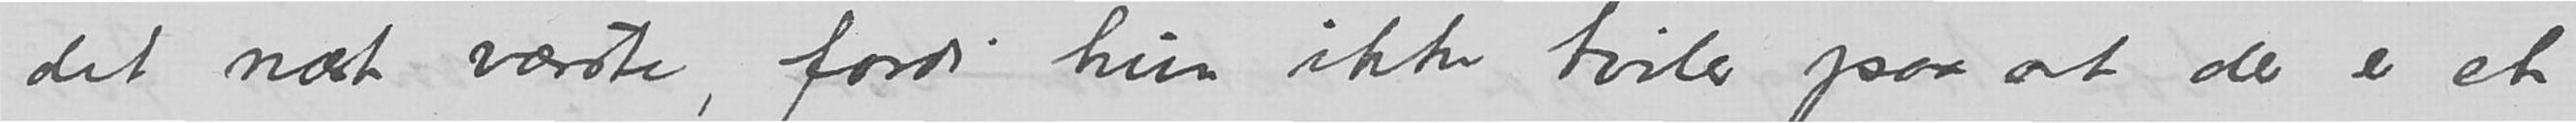det næst værste, fordi hun ikke tviler paa at der er et
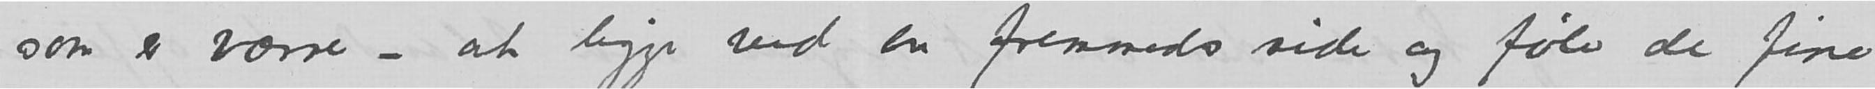som er værre - at ligge ved en fremmeds side og føle de fine
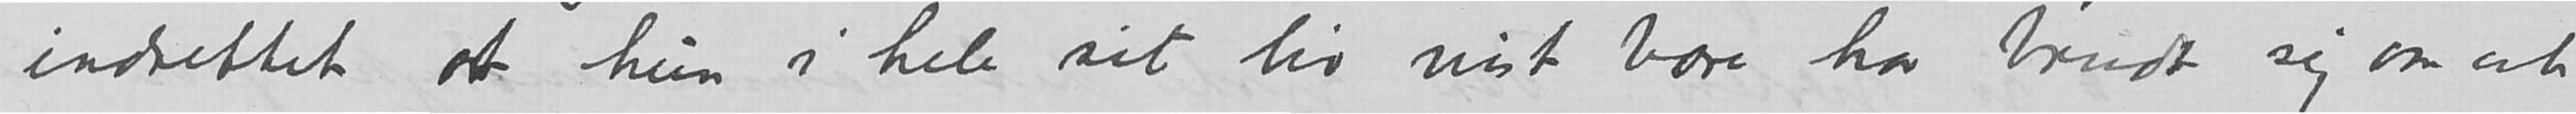indrettet at hun i hele sit liv vist bare har brudt sig om at
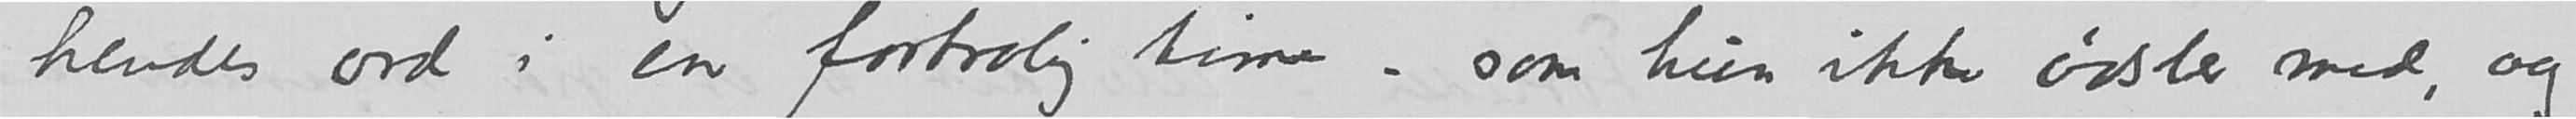hendes ord i en fortrolig time - som hun ikke ødsler med, og
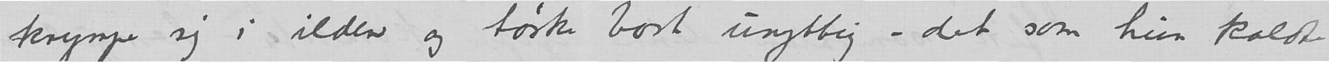krympe sig i ilden og tørke bort unyttig - det som hun kaldte
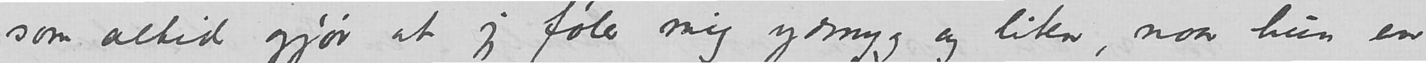som altid gjør at jeg føler mig ydmyg og liten, naar hun en
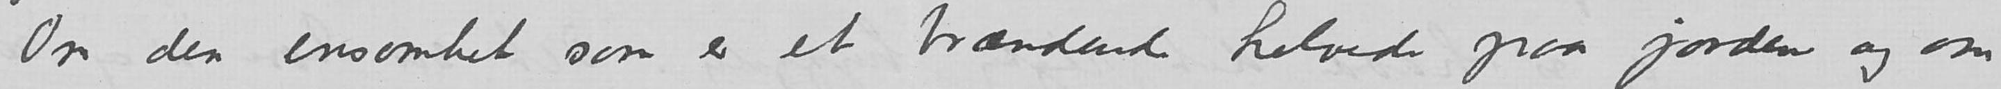Om den ensomhet som er et brændende helvede paa jorden og
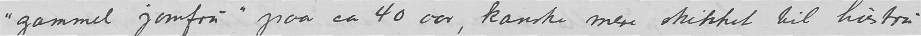«gammel jomfru» paa ca 40 aar, kanskje mere skikket til hustru
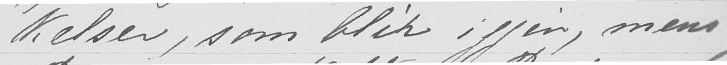kelser, som blir igjen, mens
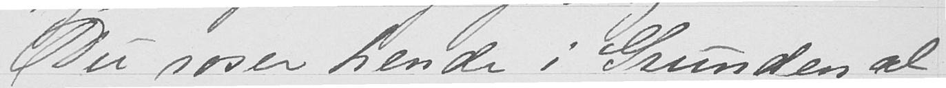Du roser hende i Grunden al-
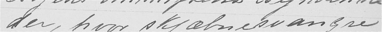der, hvor skjæbnesvangre
